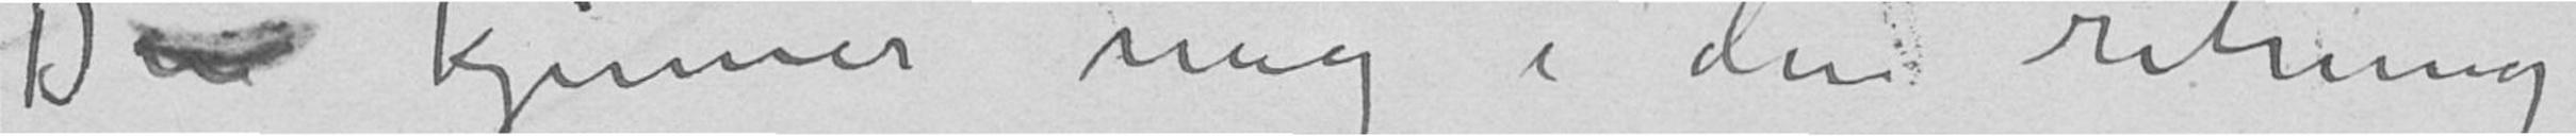Du kjenner mig i den retning
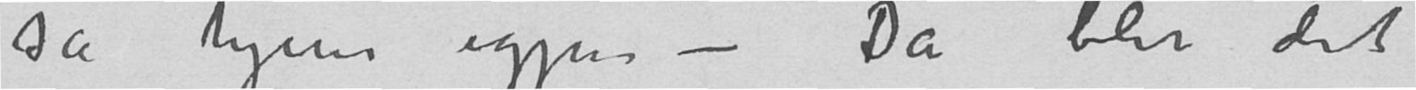så hjem igjen – Da blir det
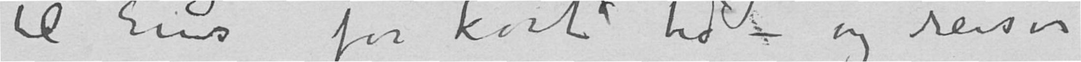til Ems for kort tid – og reiser
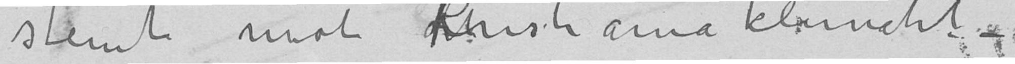stemt mot Kristiania klimatet –
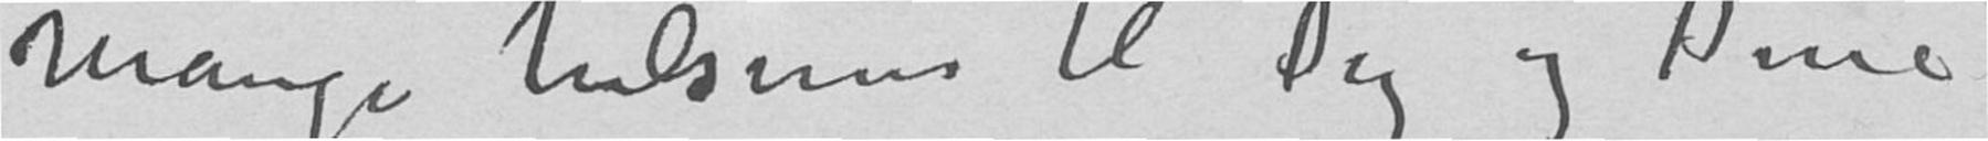Mange hilsener til Dig og Dine
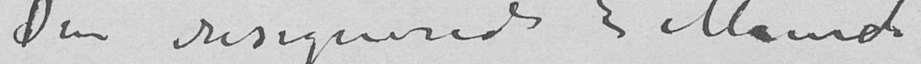Din resignerede E Munch
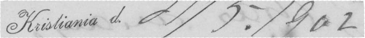Kristiania 29/5-1902
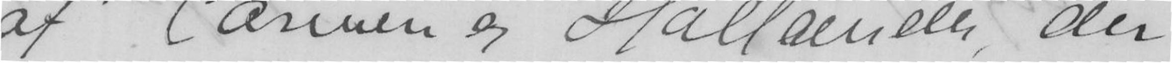af Carmen og Hollænder, der
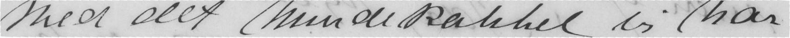Med det hundekobbel vi har
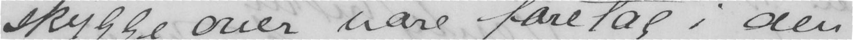skygge over vore foretag i den
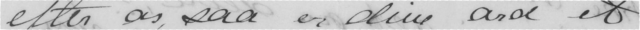efter os, saa er dine ord et
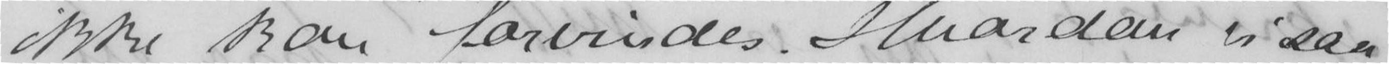ikke kan forsvinde. Hvordan vi saa
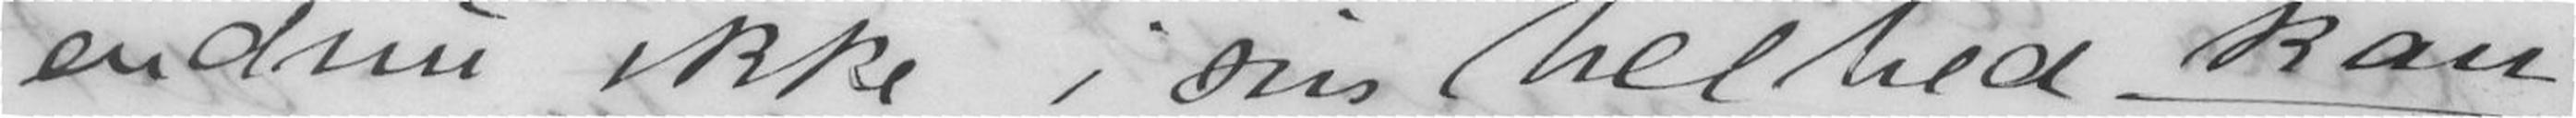endnu ikke i sin helhed kan
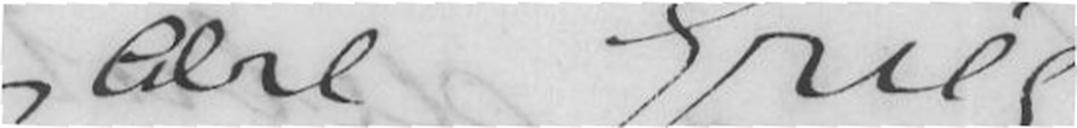Kjære Grieg.
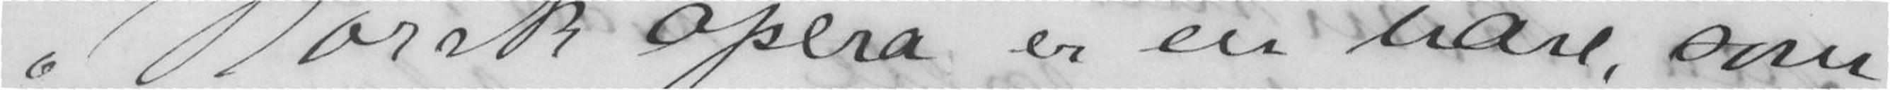Norsk opera er en vare, som
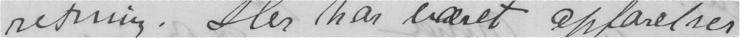retning. Her har været opførelser
